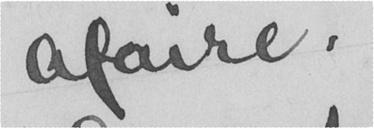afaire.
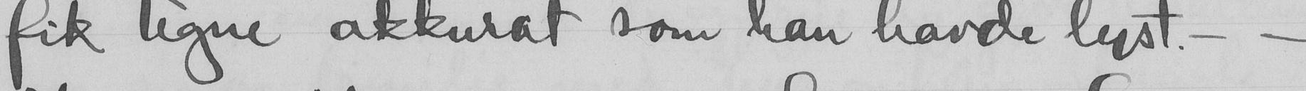fik tegne akkurat som han havde lyst. -
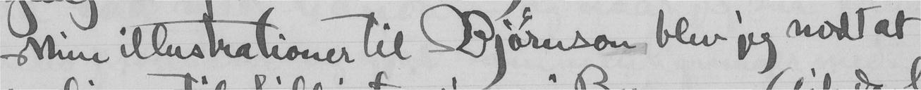Mine illustrationer til Bjørnson blev jeg nødt at
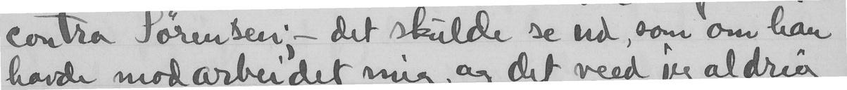contra Sørensen, - det skulde se ud, som om han
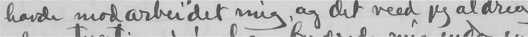havde modarbeidet mig, og det veed jeg aldrig
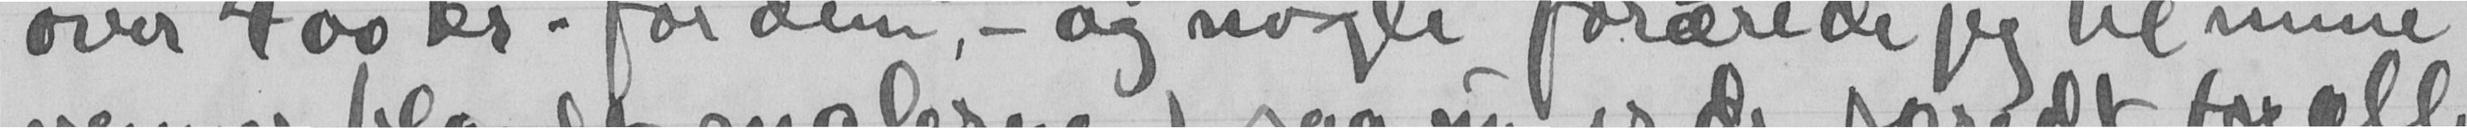over 400 kr.- for dem, - og nogle forærede jeg til mine
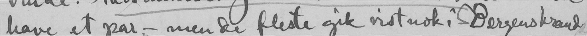have et par, - men de fleste gik vistnok i - Bergens krænds
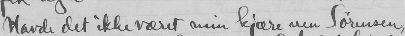Havde det ikke været min kjære ven Sørensen,
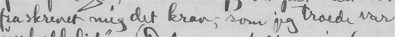fraskrevet mig det krav, som jeg troede var
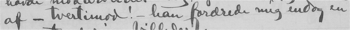af - tvertimod! - han fordrede mig endog en
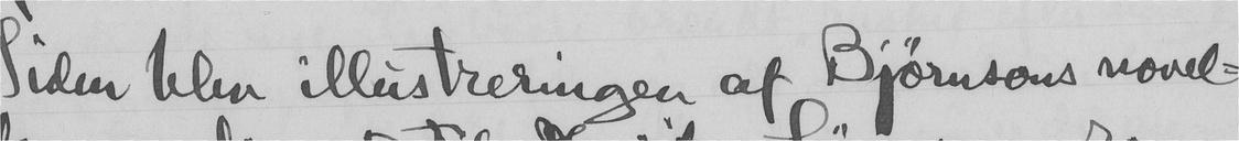Siden blev illustreringen af Bjørnsons novel-
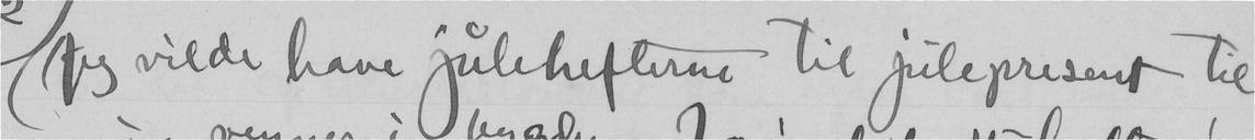(Jeg vilde have julehefterne til julepresent til
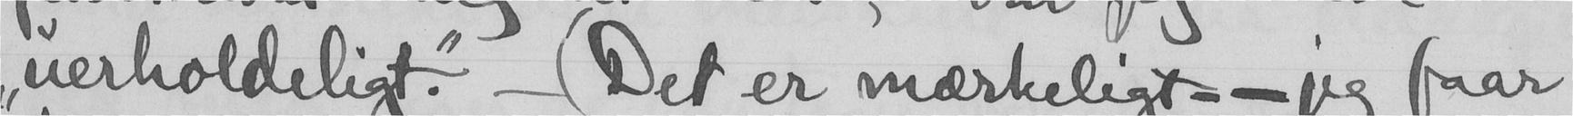"uerholdeligt". - (Det er mærkeligt. - jeg faar
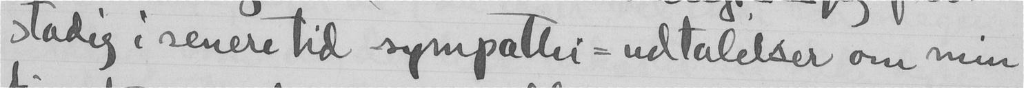stadig i senere tid sympathi - udtalelser om min
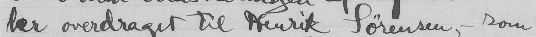her overdraget til Henrik Sørensen, - som
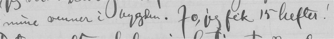mine venner i bygden. Jo, jeg fik 15 hefter!
